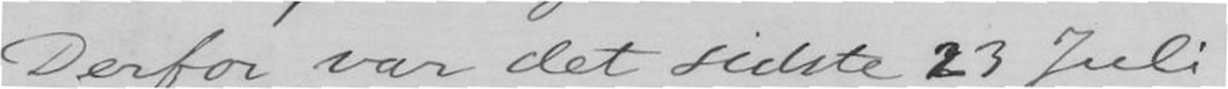Derfor var det sidste 23 Juli
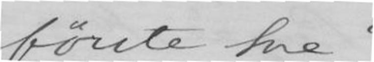første Sne.
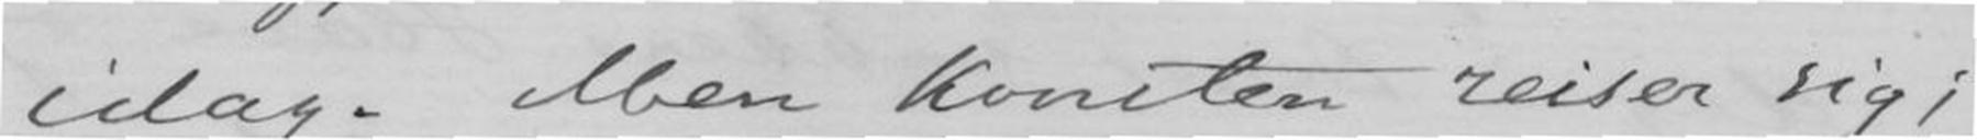idag. Men konsten reiser sig;
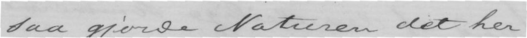saa gjorde Naturen det her
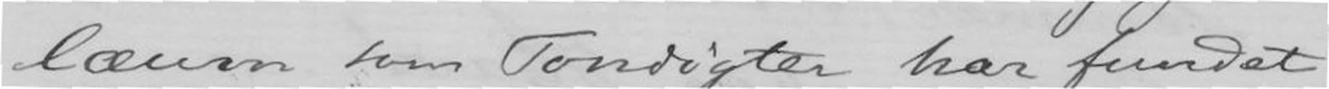læum som Tondigter har fundet
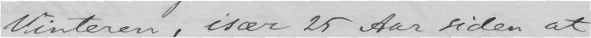Vinteren, især 25 Aar siden at
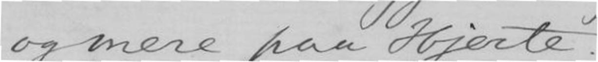og mere paa Hjerte.
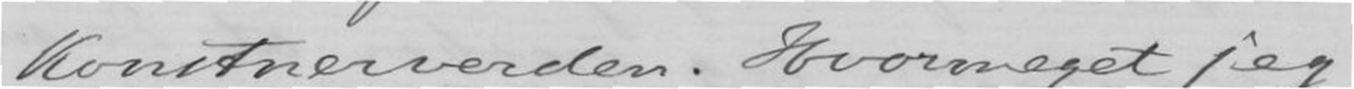Konstnerverden. Hvormeget jeg
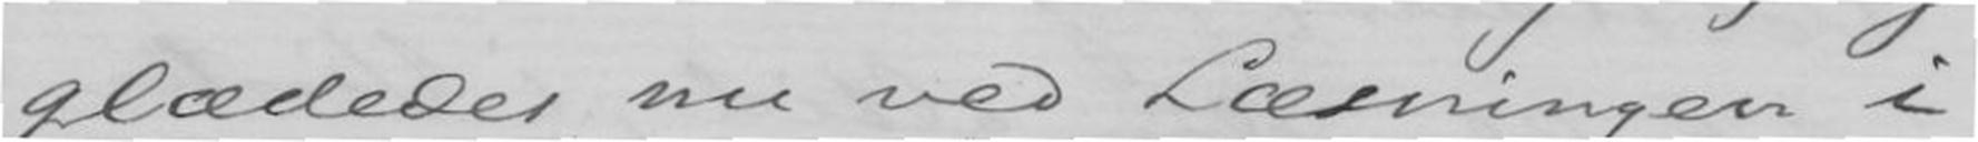glædedes nu ved Læsningen i
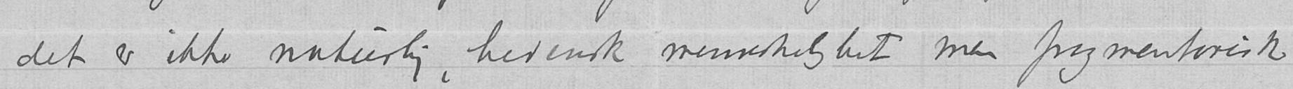det er ikke naturlig, hedensk menneskelighet men fragmentarisk
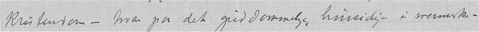kristendom – troen paa det guddommelige, hinsidige i menneske-
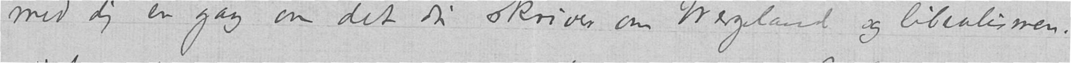med dig en gang om det du skriver om Wergeland og liberalismen.
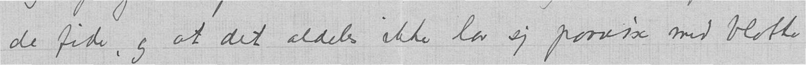de fide, og at det aldeles ikke lar sig paavise med blotte
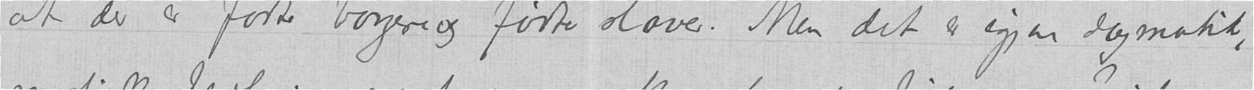at der er fødte borgere og fødte slaver. Men det er igjen dogmatik,
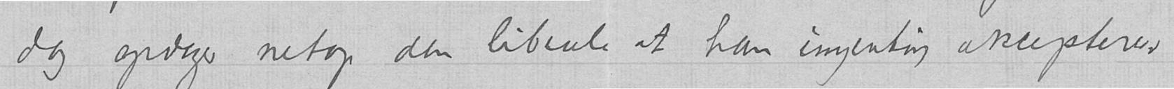dag opdager netop den liberale at han ingenting akcepterer
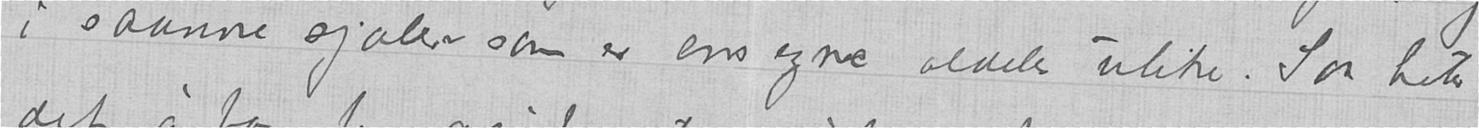i saanne sjæler som er ens egne aldeles ulike. Saa heter
